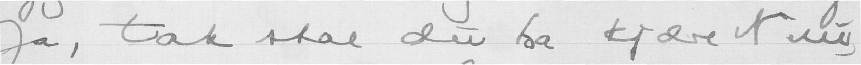Ja, tak skal du ha kjære Nini,
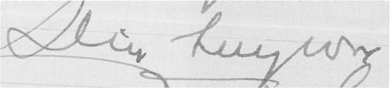Din hengivne
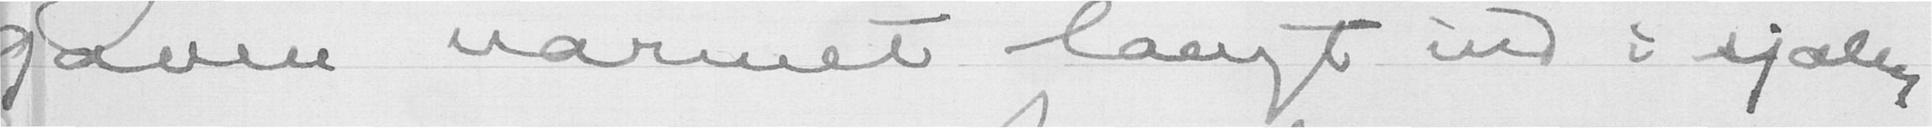gaven varmet langt ind i sjælen.
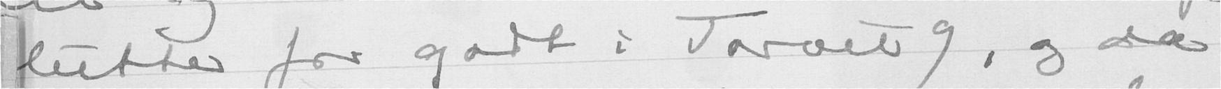lutter for godt i Torvet g, og da
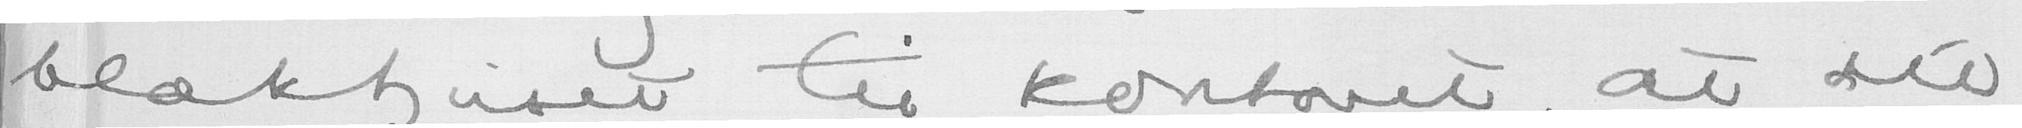bloktjaser til kontoret at du
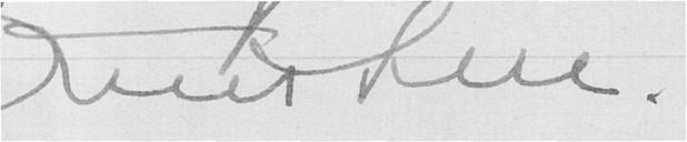lentkurl.
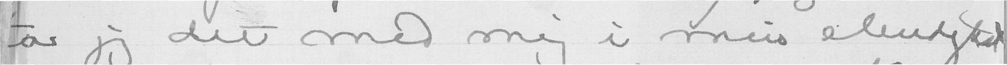tarr jeg det med mig i Son alendgtet.
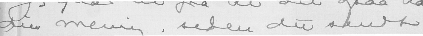med mening, siden du skudt
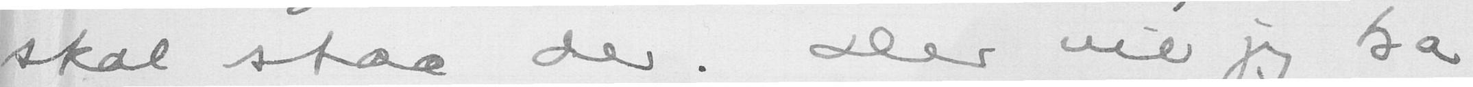skal staa der. Der vil jeg ha
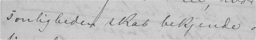sonligheden skal bekjende og
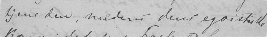tjene den, medens dens egoistiske
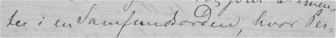ter i en Samfundsorden, hvor Per-
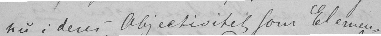nu i deres Objectivitet som Elemen-
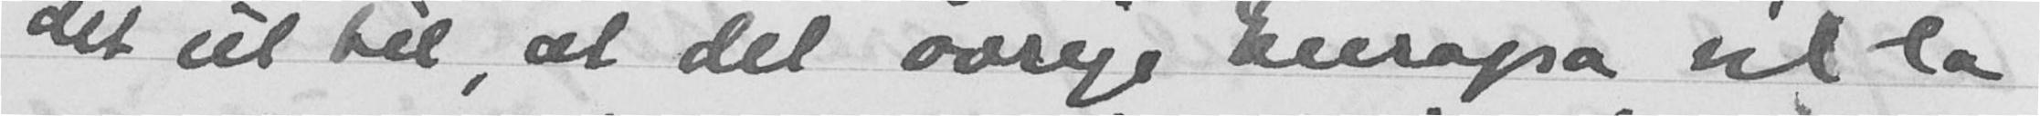det ut til, at det øvrige Europa vil la
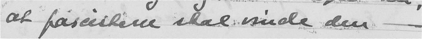at fascistene skal vinde den -.
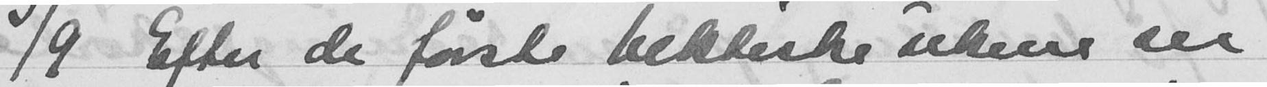3/9 Efter de første hektiske ukene ser
